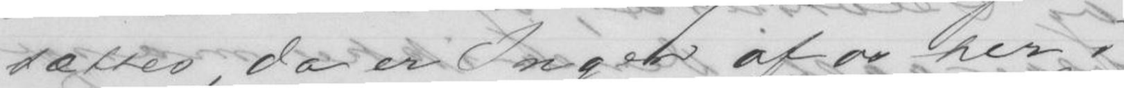sættes, da er Ingen af os her i
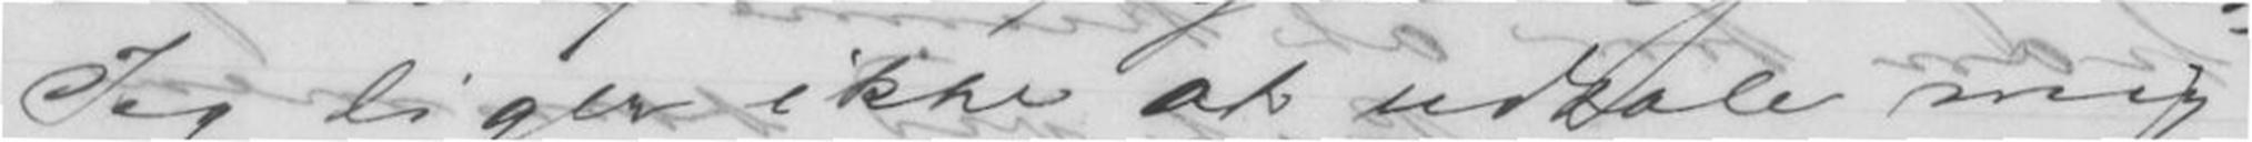Jeg liger ikke at udtale mig
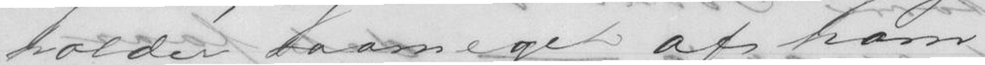holder saameget af ham
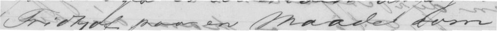Fridtjof paa en Maade som
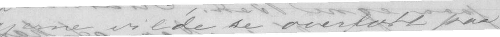gjerne vilde se overført paa
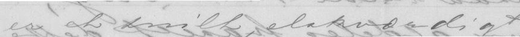er et snilt, elskværdigt
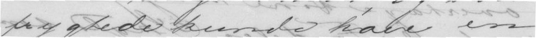frygtede kunde have en
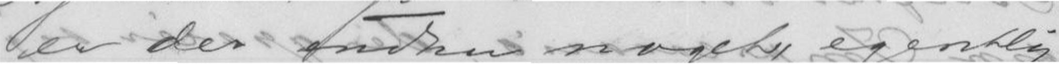er der endnu noget, egentlig
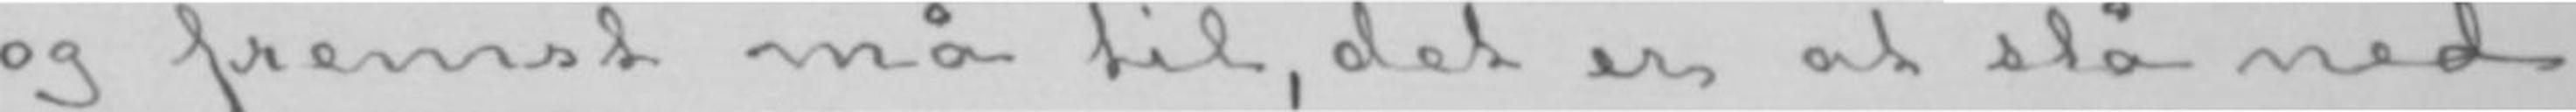og fremst må til, det er at slå ned
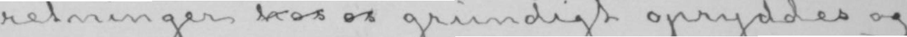retninger hos os grundigt opryddes og
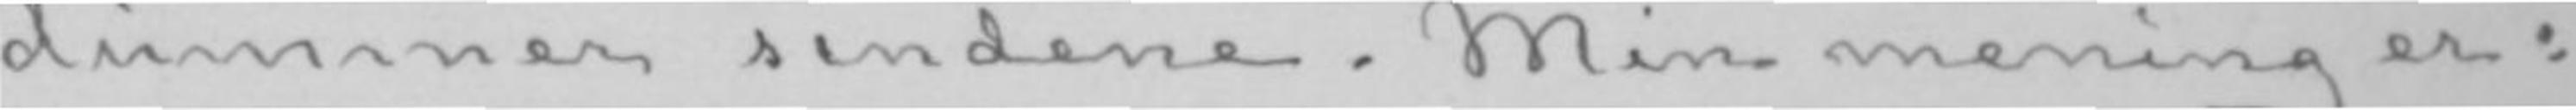dummer sindene. Min mening er:
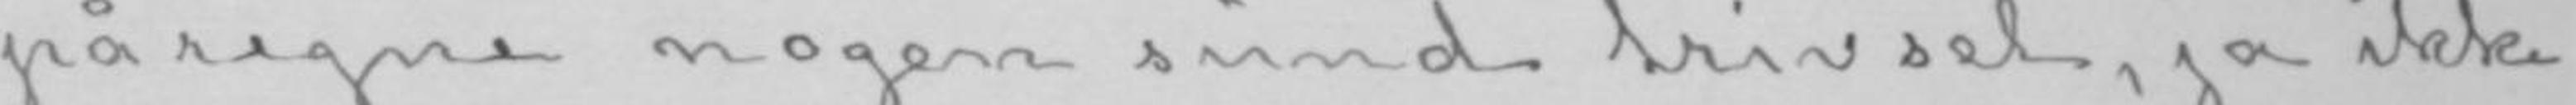påregne nogen sund trivsel, ja ikke
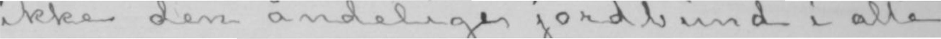ikke den åndelige jordbund i alle
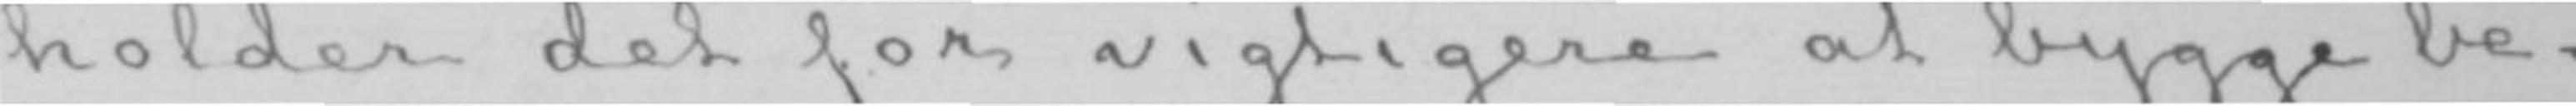holder det for vigtigere at bygge be-
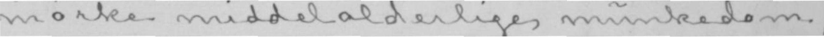mørke middelalderlige munkedom,
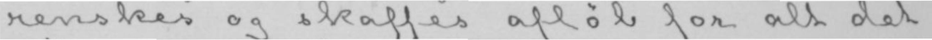renskes og skaffes afløb for alt det
